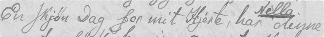En skjøn Dag for mit Hjerte, har denne
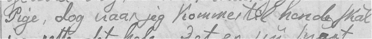Pige, dog naar jeg kommer til hende skal
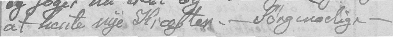at hente nye Kræfter. – Sørgmodig. –
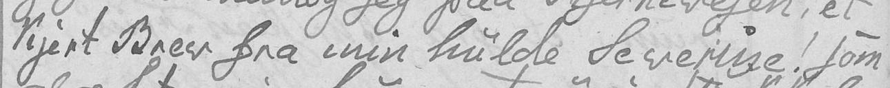kjert Brev fra min hulde Severine! som
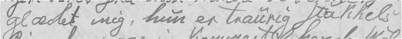glædet mig, hun er traurig stakkels
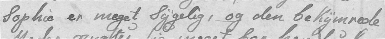Sophie er meget Sygelig, og den bekymrede
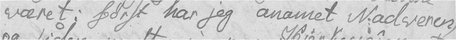været: først har jeg anamet Nadveren,
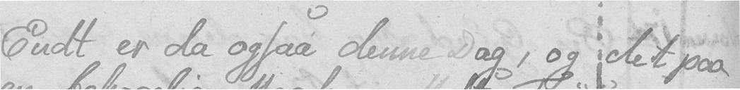Endt er da ogsaa denne Dag, og det paa
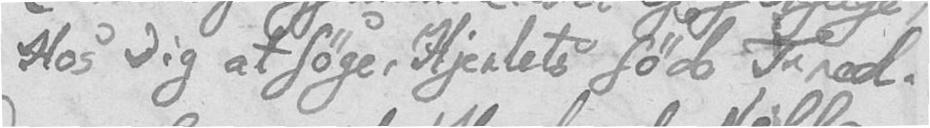Hos Dig at søge, Hjertets søde Fred!
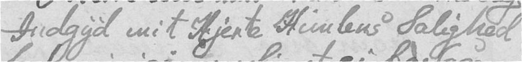Indgyd mit Hjerte Himlens Salighed
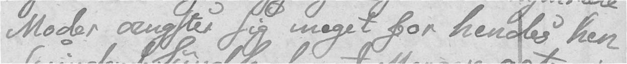Moder ængster sig meget for hendes hen
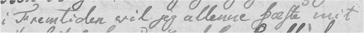i Fremtiden vil jeg allenne fæste mit
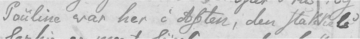Pouline var her i Aften, den stakkels
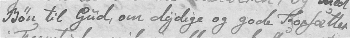Bøn til Gud, om dydige og gode Forsætter
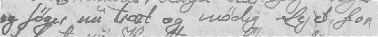og søger nu træt og mødig Lejet, for
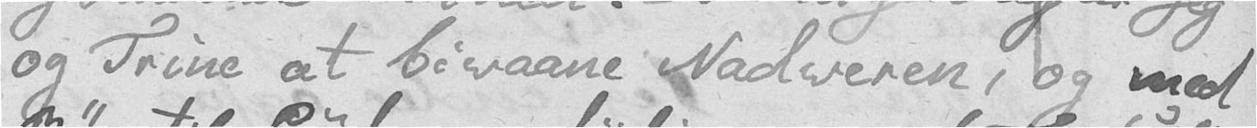og Trine at bivaane Nadveren, og med
